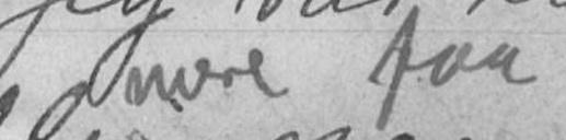mere faa
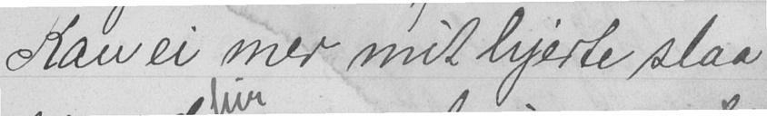Kan ei mer mit hjerte slaa
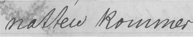natten kommer
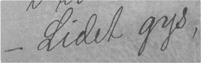- Lidet gys,
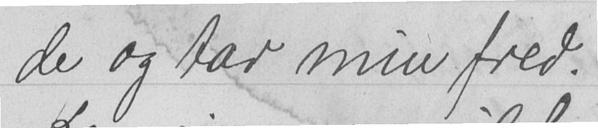de og tar min fred.
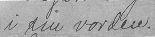i sin uorden.
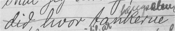did, hvor tankerne
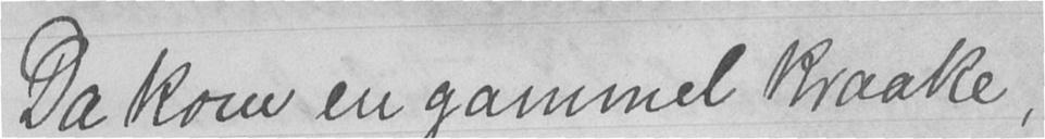Da kom en gammel kraake,
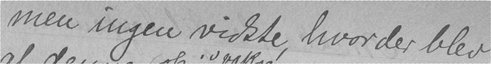men ingen vidste, hvor der blev
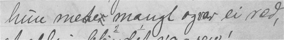hun meder mangt og var ei ræd,
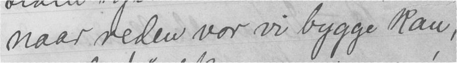naar reden vor vi bygge kan,
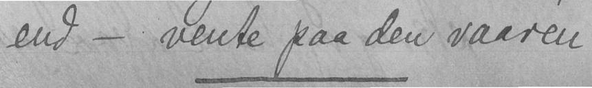end - vente paa den vaaren.
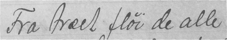Fra træet, fløi de alle
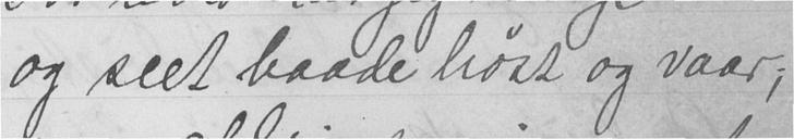og seet baade høst og vaar;
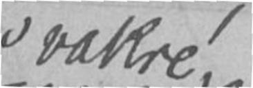'vakre'
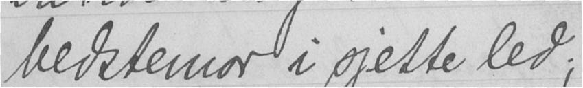bedstemor i sjette led;
